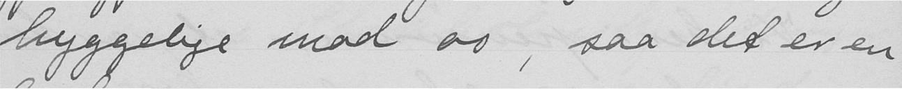hyggelige mod os, saa det er en
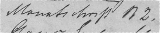Monatschrift B 2,
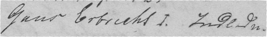Gans Erbrecht I. Indledn.
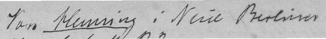Tom Hennig i Neue Berliner
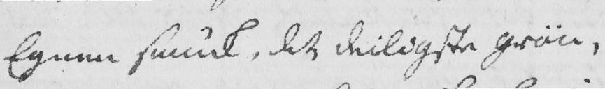Egnen smmuck, det deiligste grøn,
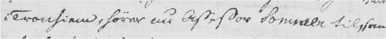i Tronhiem, hører nu Assesor Sommer til, som
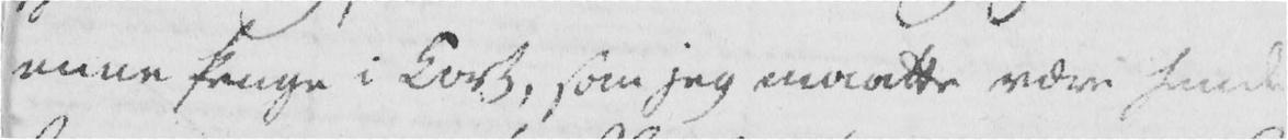mere Penge i Kort, som jeg maatte være hende
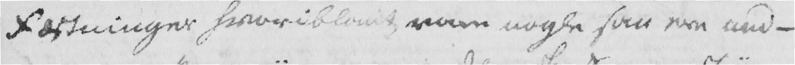Fæstninger hvoriblandt vare nogle som ere ned-
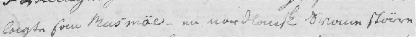lagte som Musmøe - en nordlansk Svane større
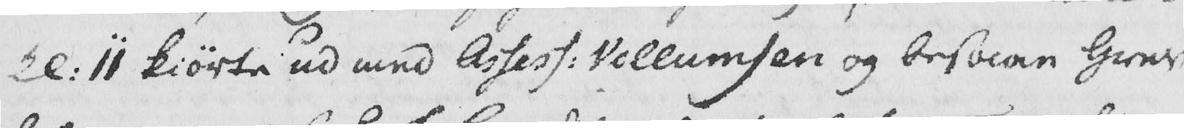kl. 11 kiørte ud med Assess. Villumsen og besaae Grev
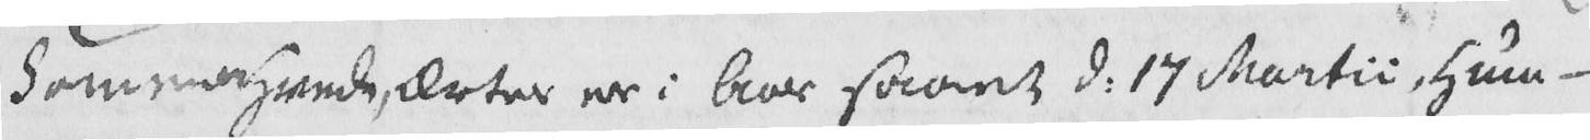Sommer-Hvete, Ærter er i Aar saaet d. 17 Martii, Hum-
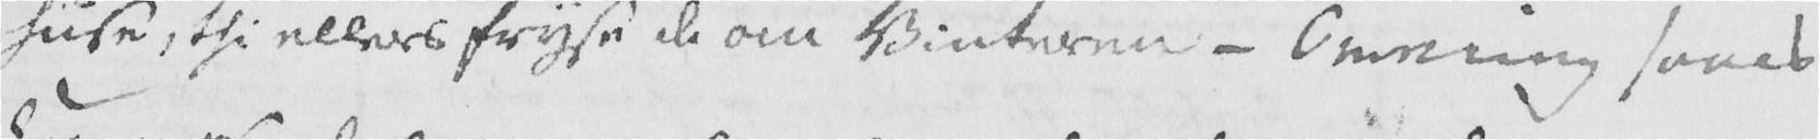huse, thi ellers fryse de om Vinteren - Omkring saaes
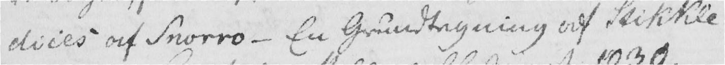dices af Snorro - En Grundtegning af Stikkle-
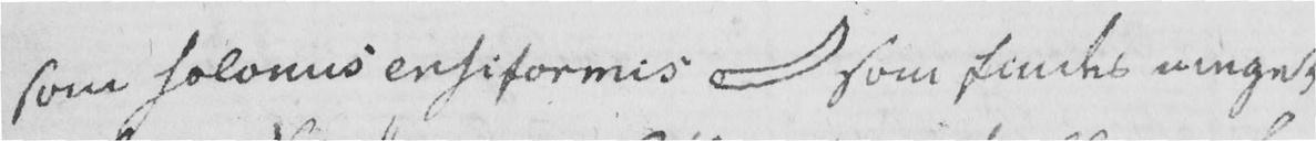som solonus ensiformis  som findes meget
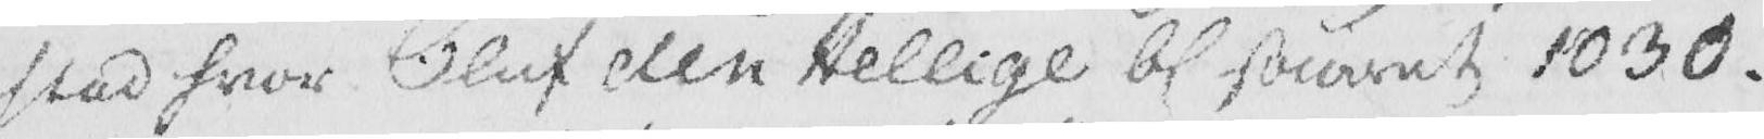stad hvor Oluf den Hellige bl saaret 1030.
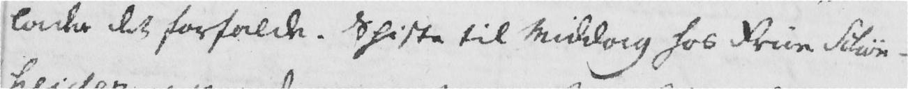lader det forfalde. Spiste til Middag hos Frue Schøn-
